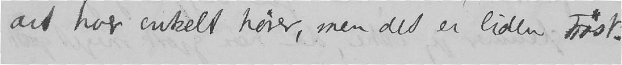art hver enkelt hører, men det er liden trøst.
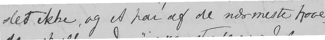slet ikke, og et par af de nærmeste hove-
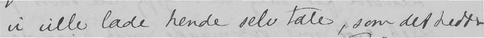vi ville lade hende selv tale, som det hedder
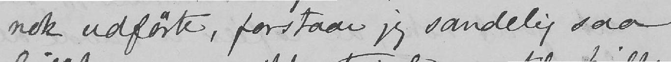nok udførte, forstaar jeg sandelig saa
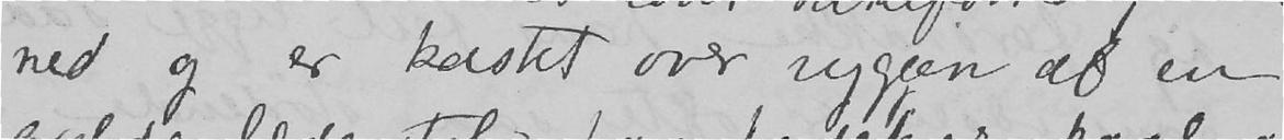ned og er kastet over ryggen af en
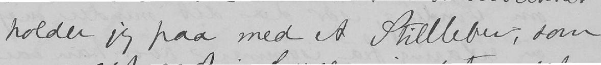holder jeg paa med et Stilleben, som
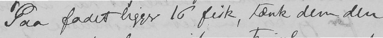Paa fadet ligger 16 fisk, tænk dem den
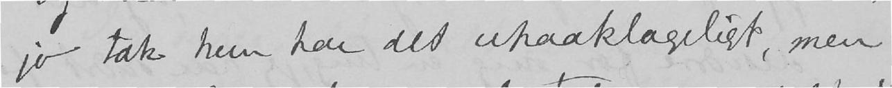jo tak hun har det upaaklageligt, men
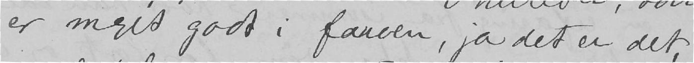er meget godt i farven, ja det er det,
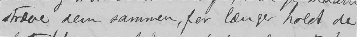stræve dem sammen, for længer holdt de
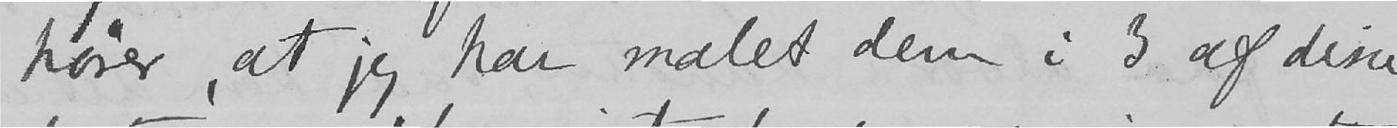hører, at jeg har malet dem i 3 af disse
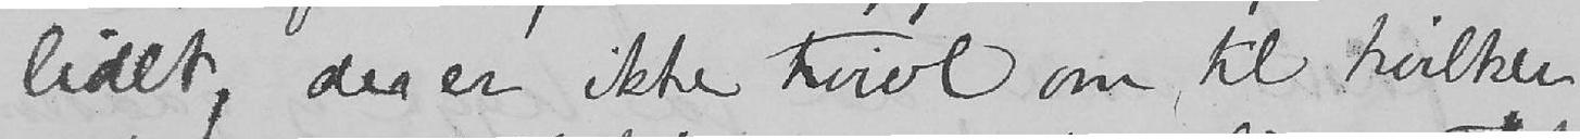lidet, der er ikke tvivl om til hvilken
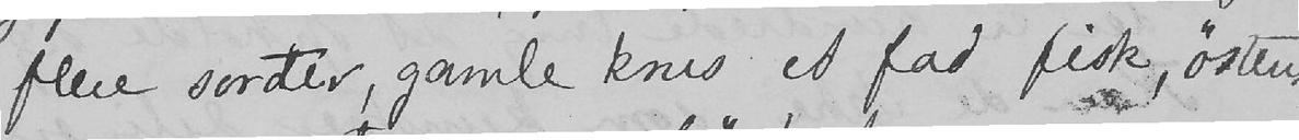flere sorter, gamle krus et fad fisk, østers
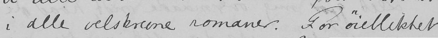i alle velskrevne romaner. For øieblikket
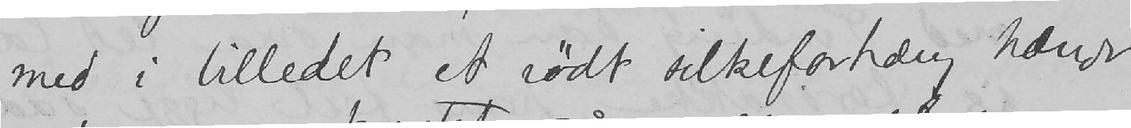med i billedet et rødt silkeforhæng hænger
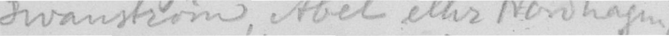Swanstrøm, Abel eller Nordhagen.)
